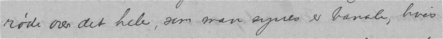røde over det hele, som man synes er banale, hvis
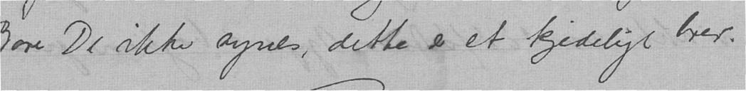Bare De ikke synes, dette er et kjedeligt brev.
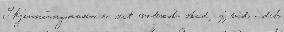Skjennungsaasen er det vakreste sted, jeg ved - det
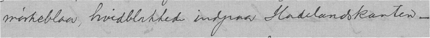mørkeblaa, hvidblakkede indpaa Hadelandskanten -
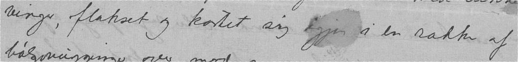vinger, flakset og kastet sig igjen i en rakke af
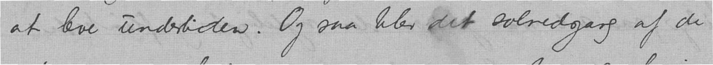at leve undertiden. Og saa blev det solnedgang af de
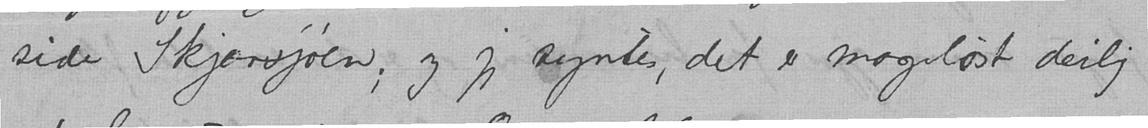side Skjærsjøen; og jeg syntes, det er mageløst deilig
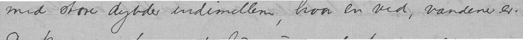med store dybder indimellem, hvor en ved, vandene er.
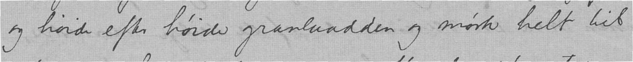og høide efter høide granlaadden og mørk helt til
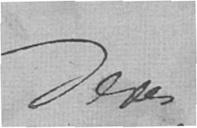Deres
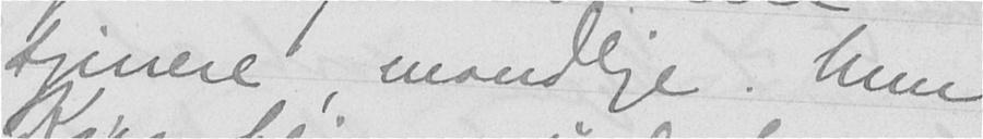tjenere, mandlige. Men
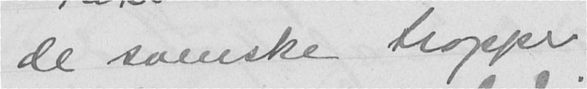de svenske tropper
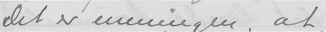det er meningen, at
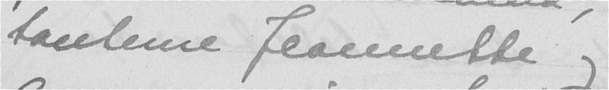tanterne Jeannette og
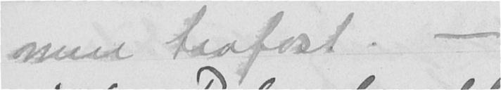men trofast. -
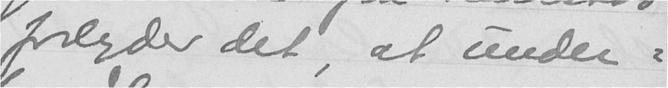forlyder det, at under-
